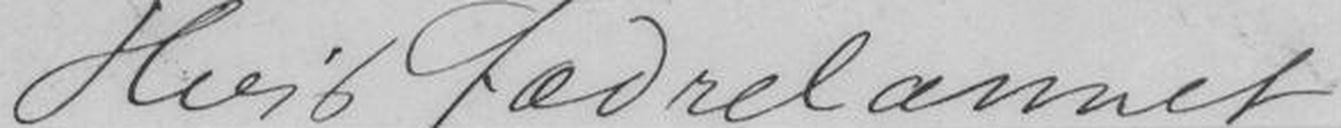Hvis fædrelannet
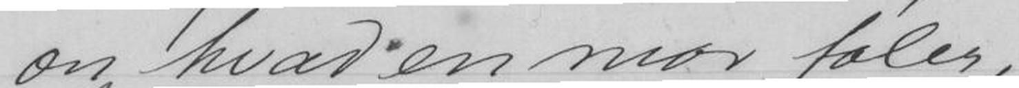æn hvad en mor føler,
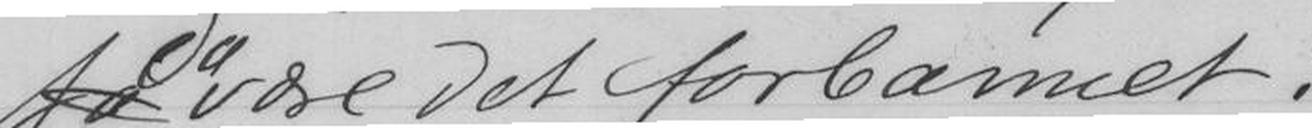så da være det forbannet!
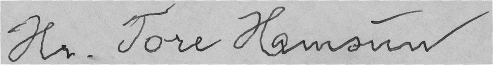Hr. Tore Hamsun
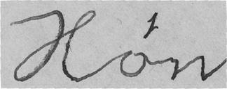Høn
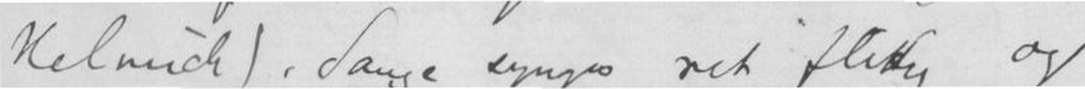Helmich). Sange synges ret flittig og
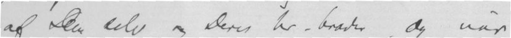af Dem selv og Deres hr. broder, og når
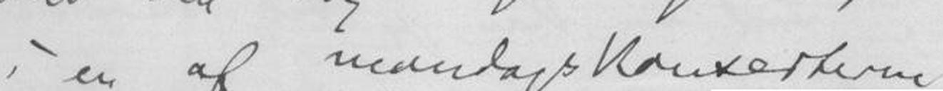i en af mandagskonserterene
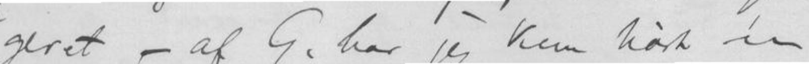geret - af G. har jeg kun hørt én
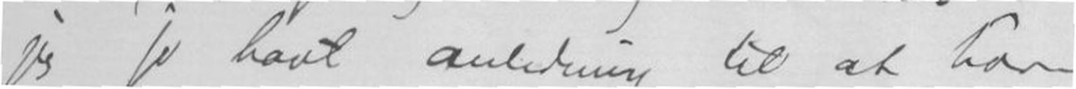jeg jo havt anledning til at haves
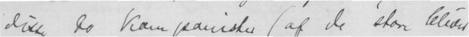disse to komponister (af de store bliveR
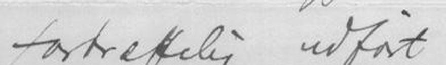fortræffelig udført.
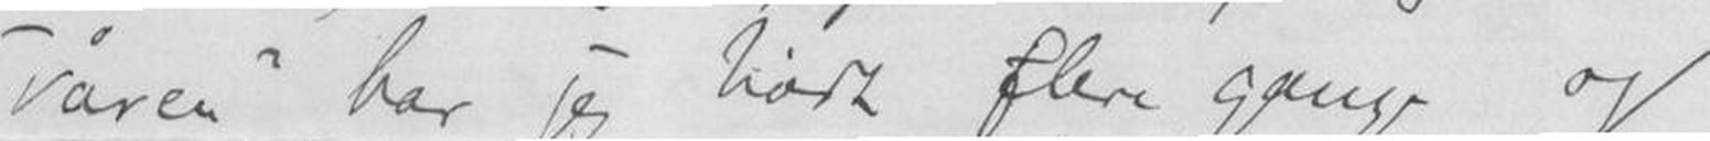"våren" har jeg hørt flere gange og
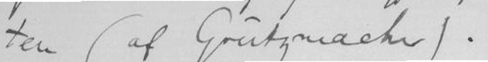ten (af Grützmacher).
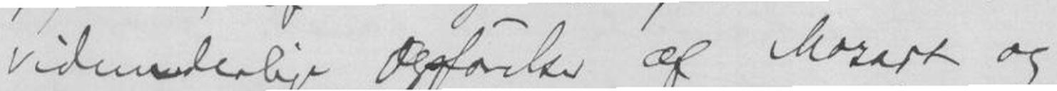vidunderlige Opførelser af Mozart og
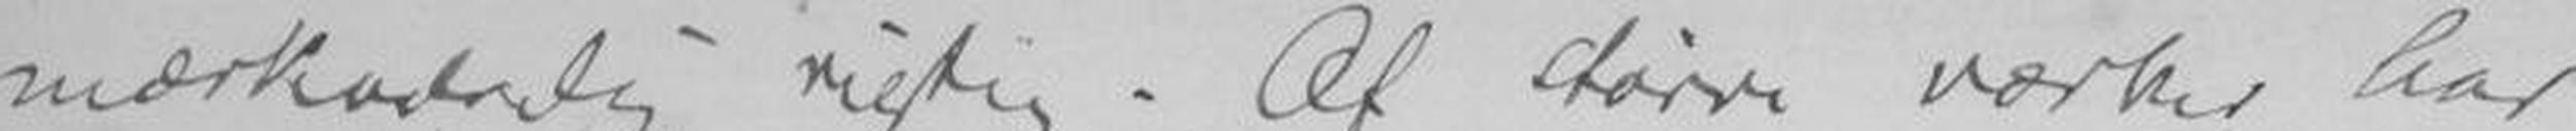mærkværdig rigtig. Af større værker har
